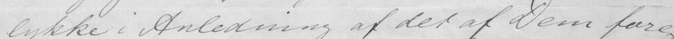lykke i Anledning af det af Dem fore-
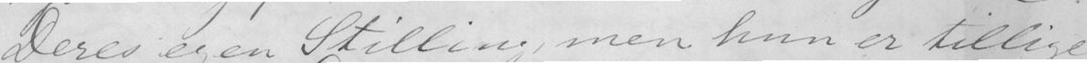Deres egen Stilling, men hun er tillige
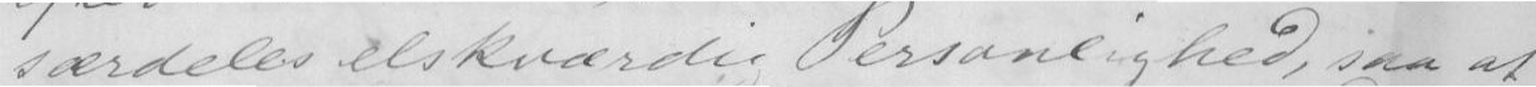særdeles elskværdig Personlighed, saa at
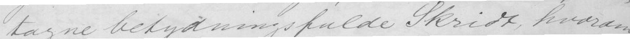tagne betydningsfulde Skridt hvorom
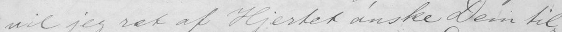vil jeg ret af Hjertet ønske Dem til-
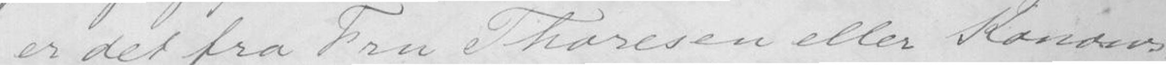er det fra Fru Thoresen eller Konows.
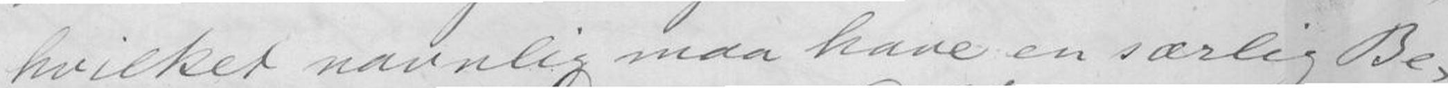hvilket navnlig maa have en særlig Be-
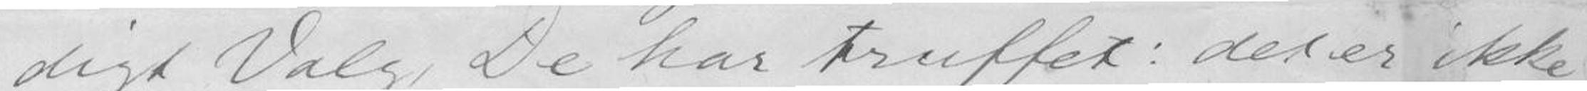digt Valg, De har truffet: det er ikke
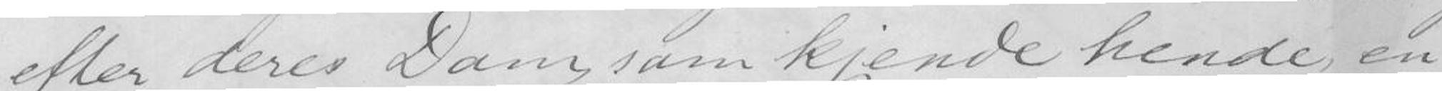efter deres Dom, som kjende hende, en
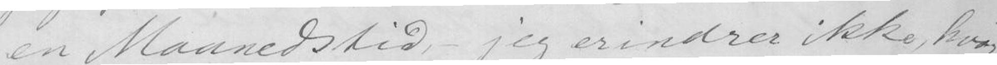en Maanedstid, - jeg erindrer ikke, hvor-
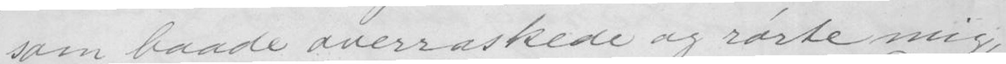som baade overraskede og rørte mig,
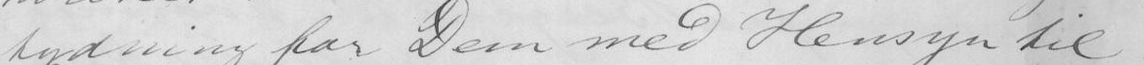tydning for Dem med Hensyn til
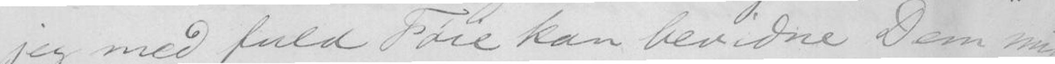jeg med fuld Føie kan bevidne Dem min
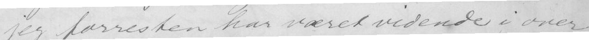jeg forresten har været vidende i over
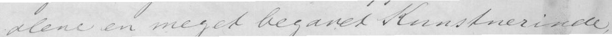alene en meget begavet Kunstnerinde
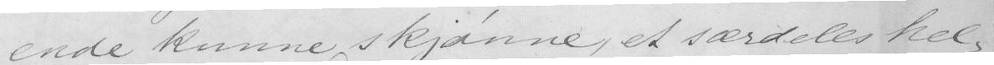ende kunne skjønne, et særdeles hel-
